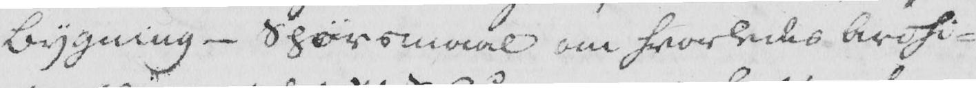Bygning - Spørsmaal om hvorledes Archi-
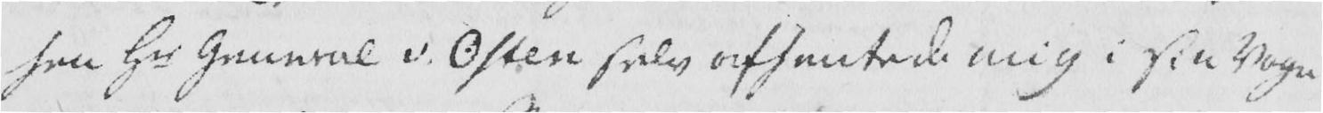hen Hr General v. Osten selv afhentede mig i sin Vogn.
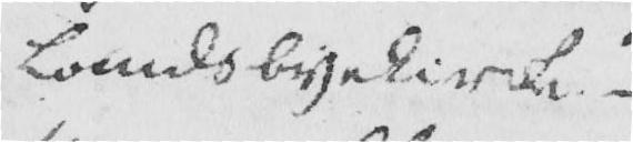Landsbyekirke. -
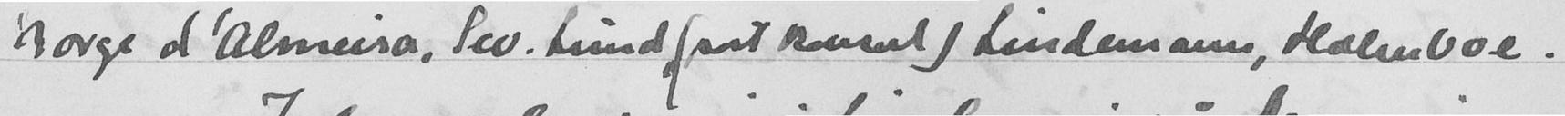Norge d'Alvnira lev. Lund(port konsul) tindemann, Holmboe.
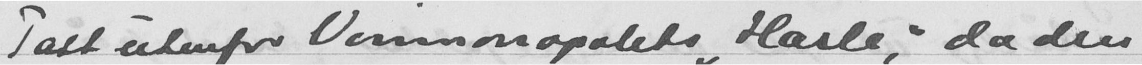Talt utenfor Vinmonopolets "Hasle" da den
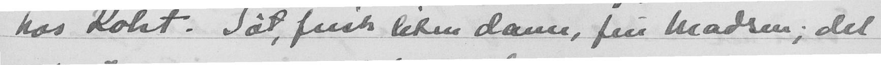hos Koht. Søt, frisk liten dame, fru Madsen; det
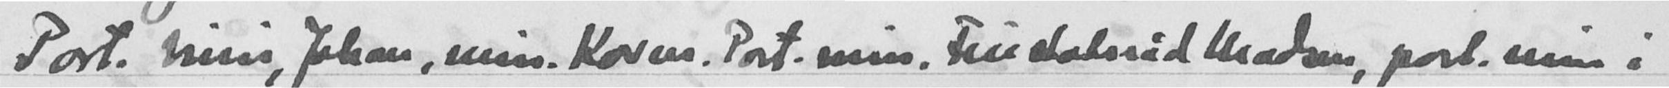Port. Nini, Johan, min. Korm. Port. min, Fru statsråd Madsen, port.min i
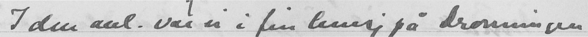I den anl. var vi i fin lunsj på dronningen
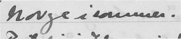Norge i sommer.
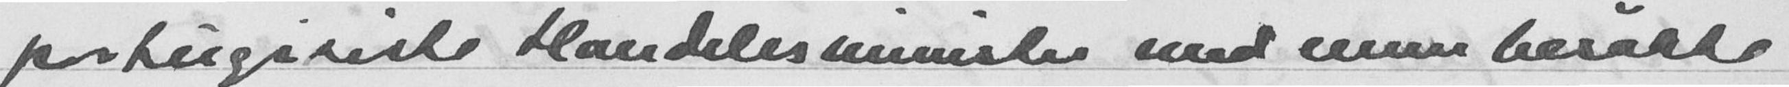portugisiske Handelesminster med menn besøkte
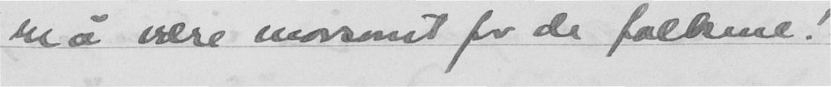må være morsomt for de folkene!
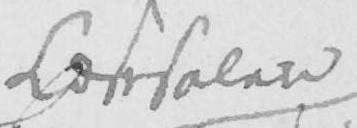Læsesalen
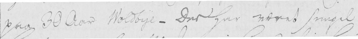paa 30 Aar Woldbye - Der har været simpel
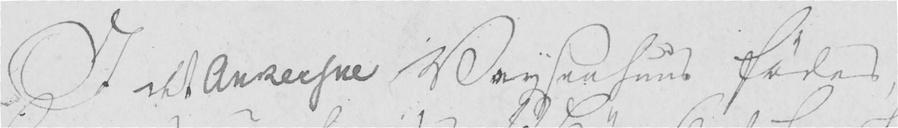I det Ankerske Vaysenhuus fødes,
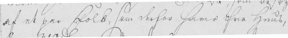af et par Folk, som derfor haver frie Huus,
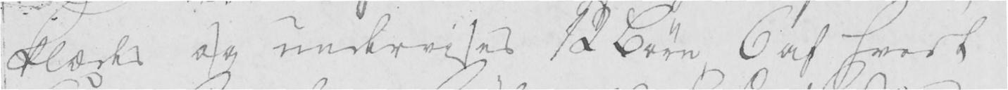klædes og undervises 12 Børn, 6 af hvert
